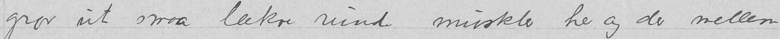gror ut smaa lækre runde muskler her og der mellem
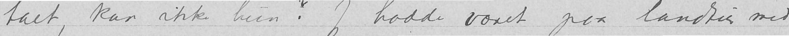talt, kan ikke hun? Jeg hadde været paa landtur med
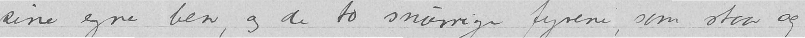sine egne ben, og de to snurrige fyrene, som staar og
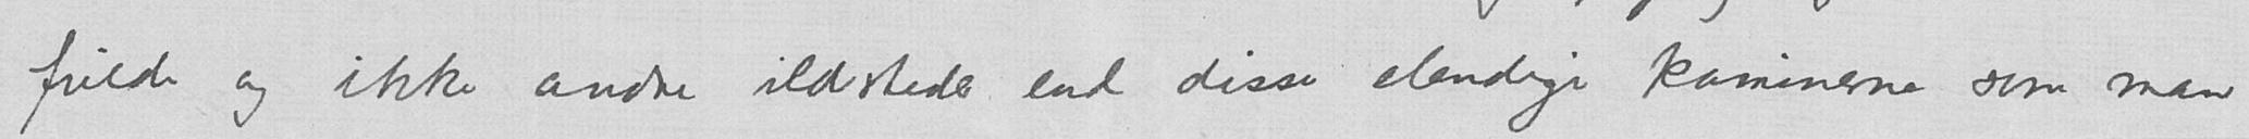fulde og ikke andre ildsteder end disse elendige kaminerne som man
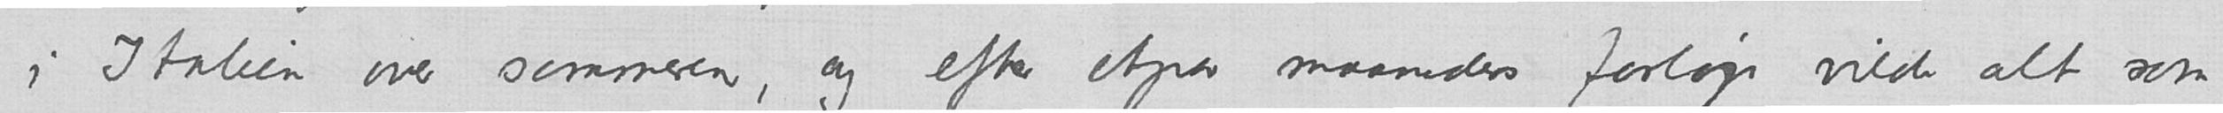i Italien over sommeren, og efter etpar maaneders forløp vilde alt som
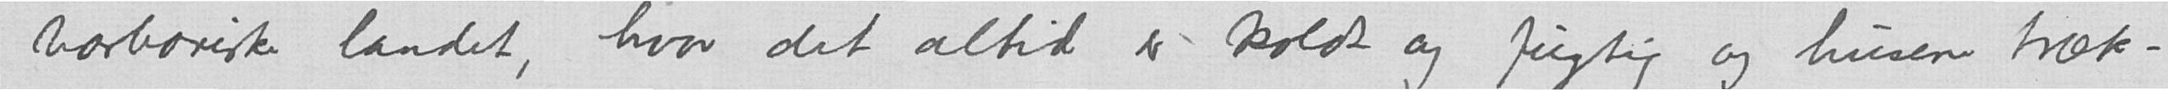barbariske landet, hvor det altid er koldt og fugtig og husene træk-
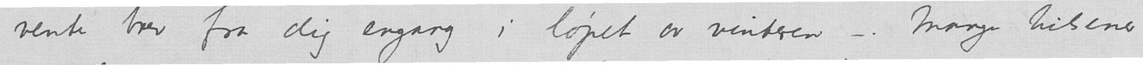vente brev fra dig engang i løpet av vinteren -. Mange hilser
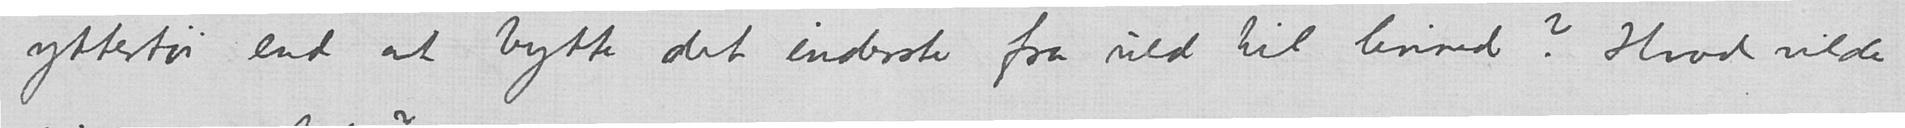yttertøi end at bytte det inderste fra uld til linned? Hvad vilde
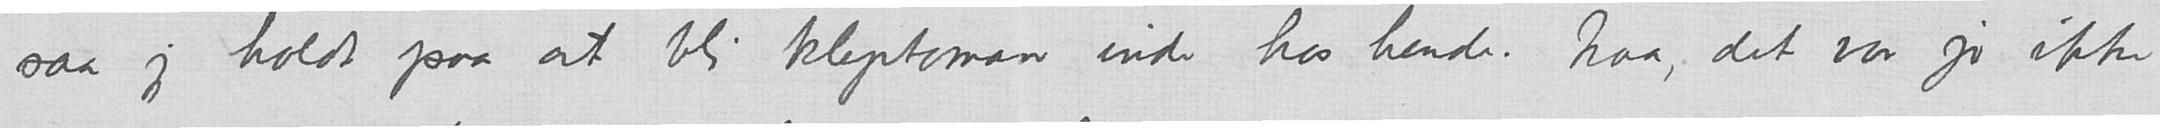saa jeg holdt paa at bli kleptoman inde hos hende. Naa, det var jo ikke
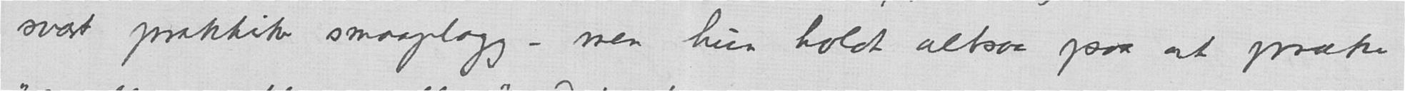svært praktiske smaaplagg - men hun holdt altsaa paa at præke
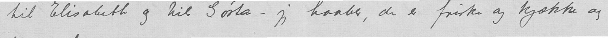til Elisabeth og til Gøsta - jeg haaber, de er friske og kjække og
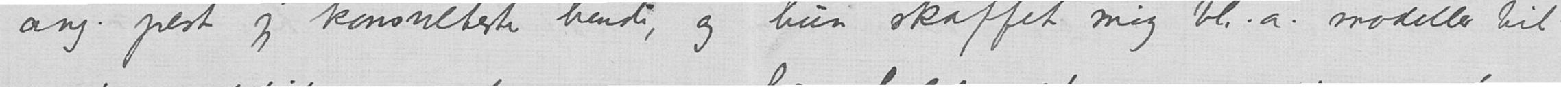ang. pest jeg konsulterte hende, og hun skaffet mig bl.a. modeller til
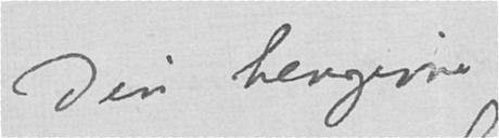Din hengivne
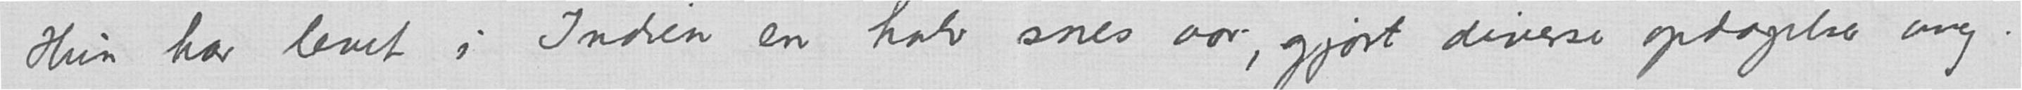Hun har levet i Indien en halv snes aar, gjort diverse opdagelser ang.
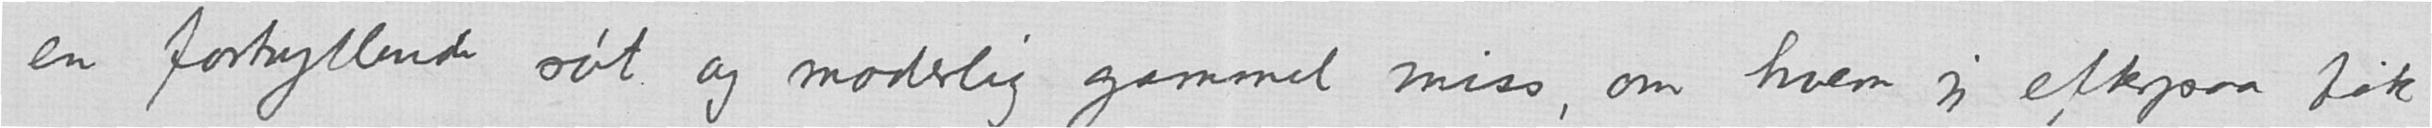en fortryllende søt og moderlig gammel miss, om hvem jeg efterpaa fik
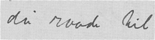du raade til?
